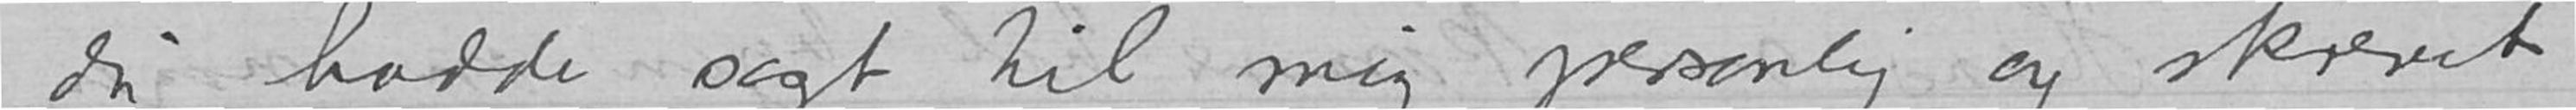du hadde sagt til mig personlig og skrevet
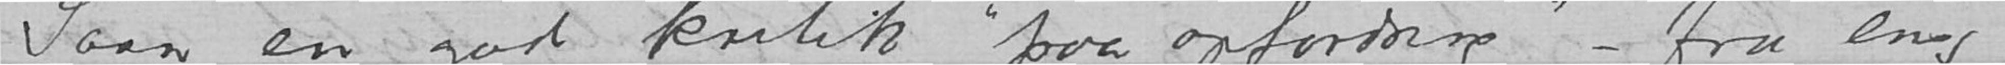Saan en god kritik «paa opfordring» – fra ens
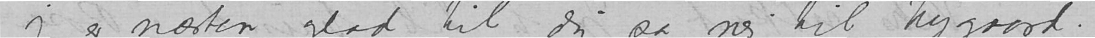jeg er næsten glad til, du sa nei til Nygaard.
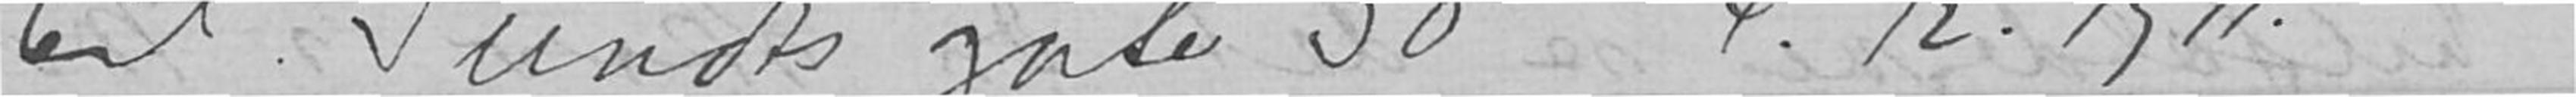Eil. Sundts gate 50 I 							4.12.1911.
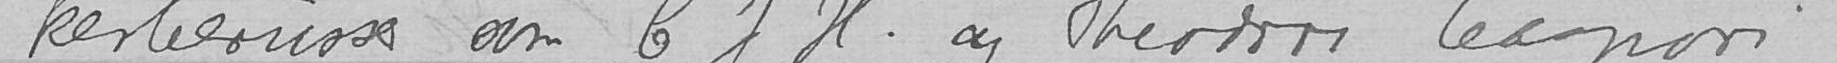kevlædrusser/karlesrisser som CJH. Og Theodor Caspari –.
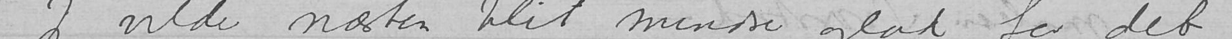Jeg vilde næsten blit mindre glad for det
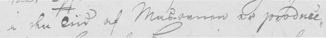i den Tiid af Mas-ovnen er produce-
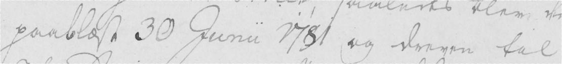paablæst 30 Junii 1781 og dreven til
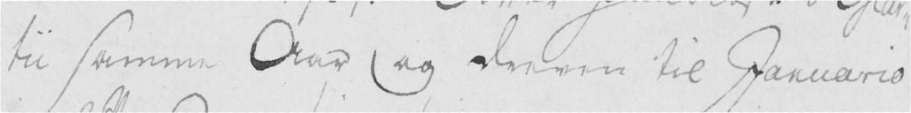tii samme Aar og dreven til Januario
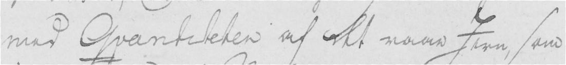med Qvantiteten af det raae Jern, som
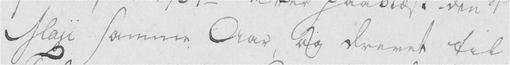Maji samme Aar, og drevet til
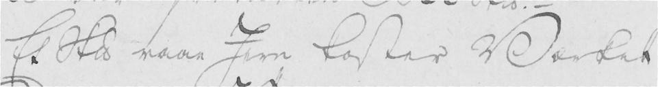Et Skpd raae Jern koster Værket
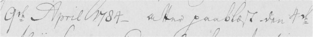9de April 1784 - atter paablæst den 4de
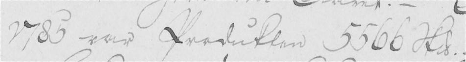1785 var Produktion 5566 Skpd. -
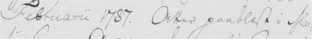Februarii 1787. Atter paablæst i Mar-
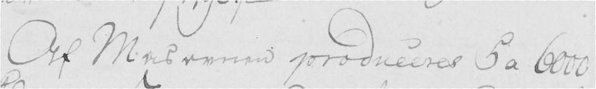Af Masovner produceres 5 a 6000
